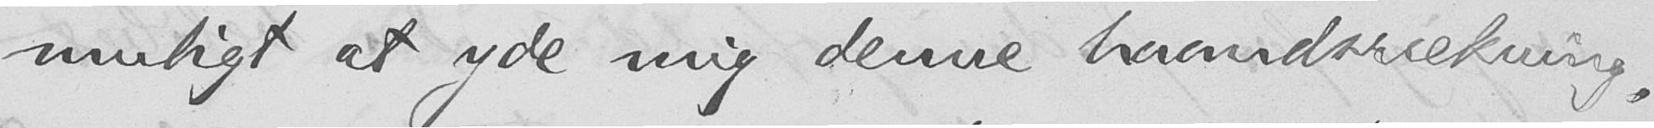muligt at yde mig denne haandsrækning,
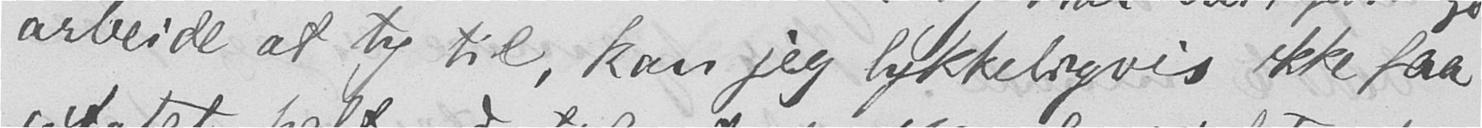arbeide at ty til, kan jeg lykkeligvis ikke faa
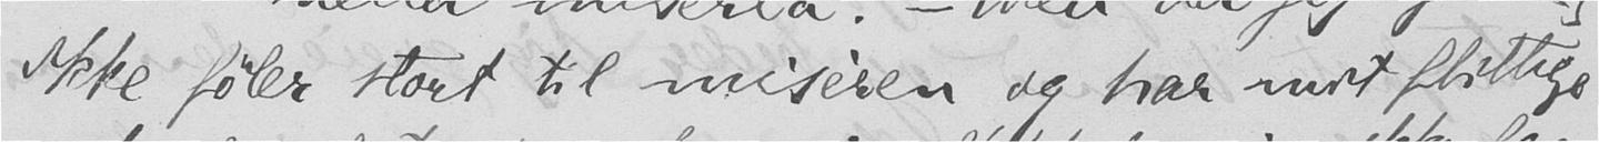ikke føler stort til miseren og har mit flittige
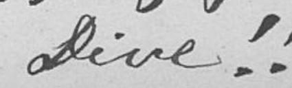Dine!!
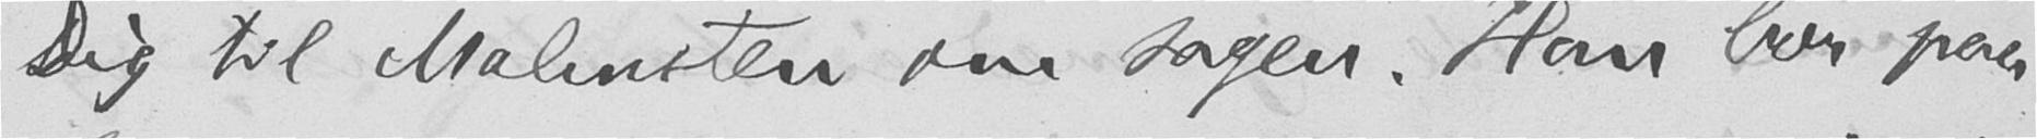Dig til Malmsten om sagen. Han bor paa
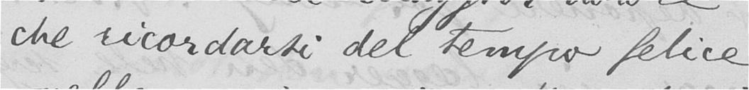che ricordarsi del tempo felice
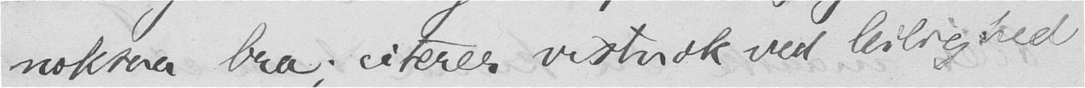noksaa bra; citerer vistnok ved leilighed
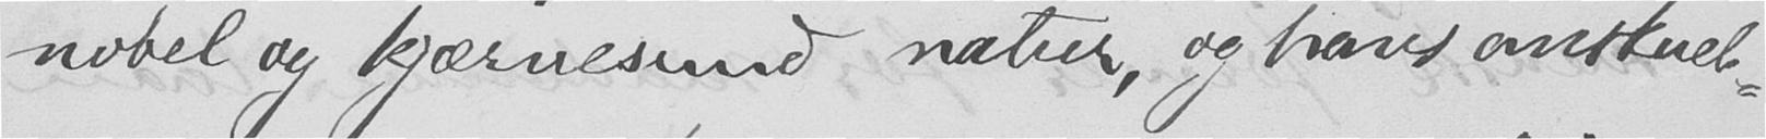nobel og kjærnesund natur, og hans anskuel-
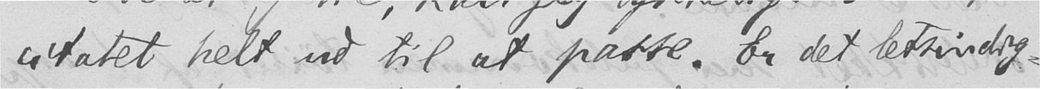citatet helt ud til at passe. Er det letsindig-
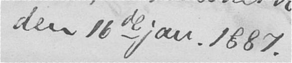den 16de jan. 1887.
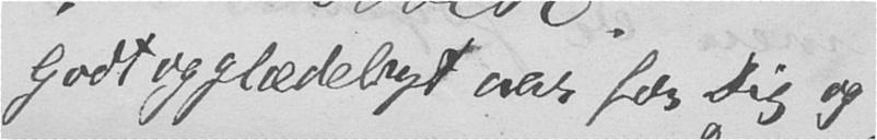Godt og glædeligt aar for Dig og
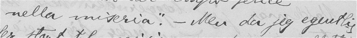nella miseria". – Men da jeg egentlig
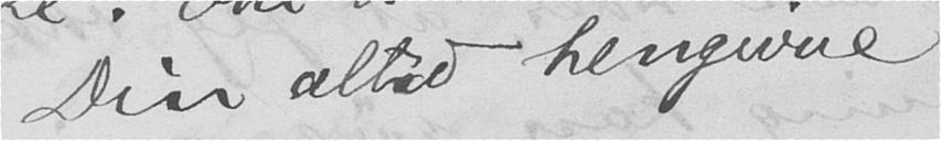Din altid hengivne
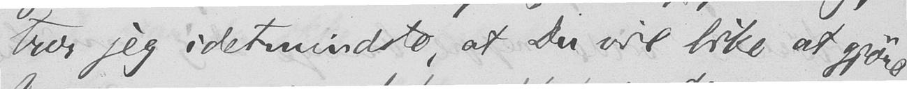tror jeg idetmindste, at Du vil like at gjøre
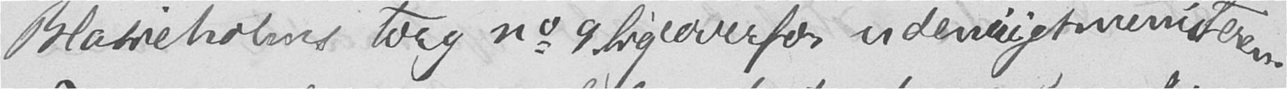Blasieholms torg no 9 ligeoverfor udenrigsministeren.
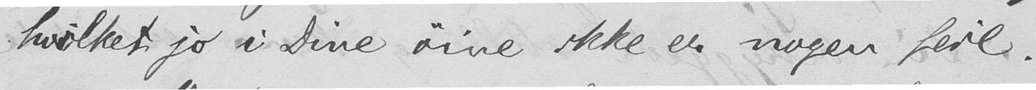hvilket jo i Dine øine ikke er nogen feil.
